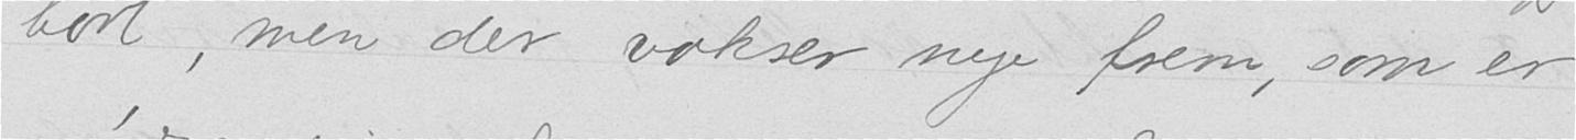bort, men der vokser nye frem, som er
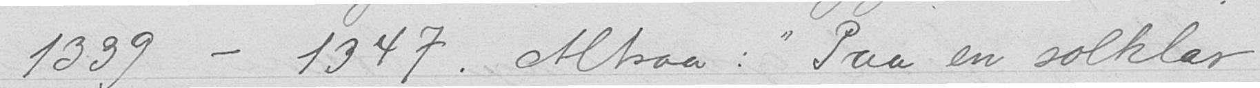1339 - 1347. Altsaa: "Paa en solklar
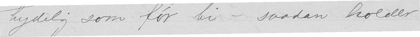tydelig som før ti - saadan holder
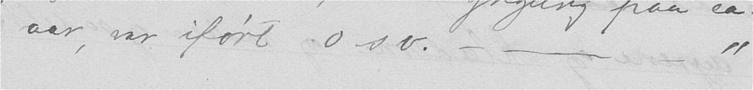aar, var iført osv. - - -"
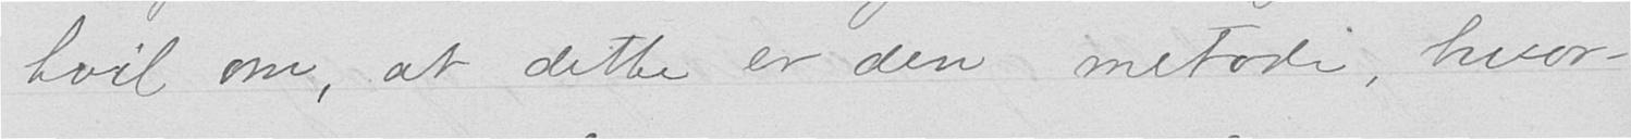tvil om, at dette er den metode, hvor-
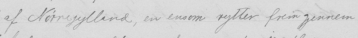af Nørrejylland, en ensom rytter frem gjennem
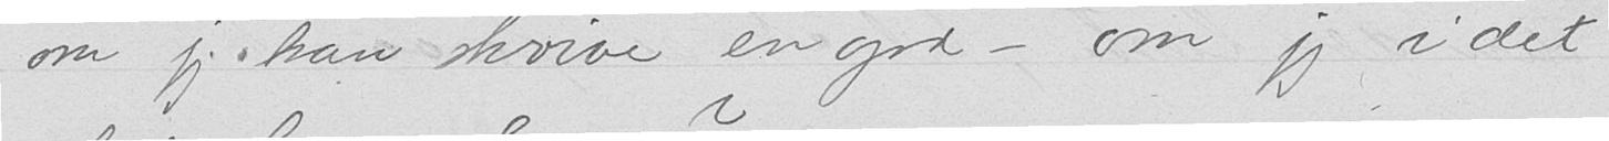om jeg kan skrive en god - om jeg i det
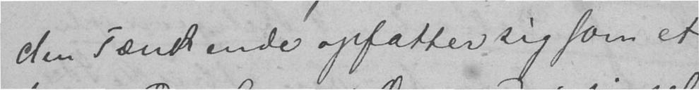den Tænkende opfatter sig som et
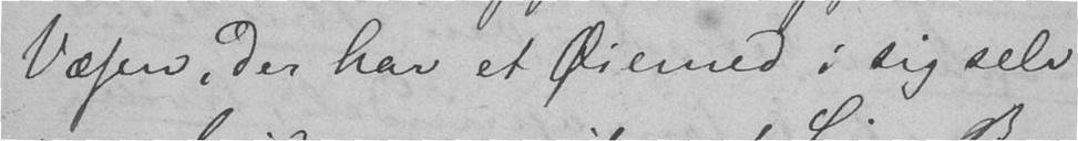Væsen, der har et Øiemed i sig selv
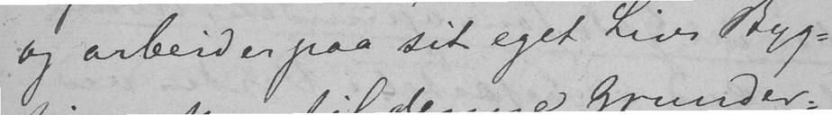og arbeider paa sit eget Livs Byg-
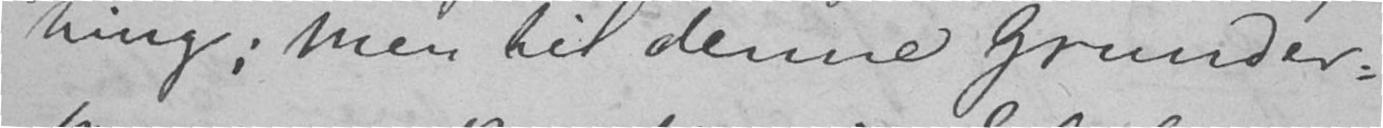ning; men til denne Grunder-
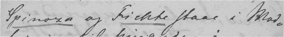Spinoza og Fichte staar i Mod-
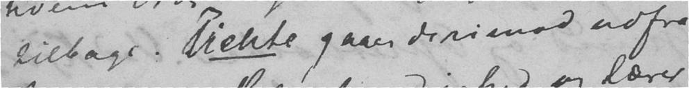tilbage. Fichte gaar derimod udfra
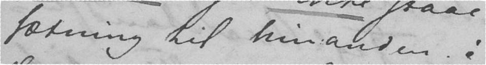sætning til hinanden:
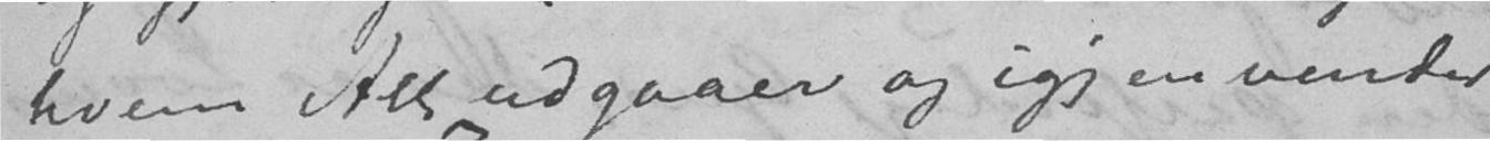hvem Alt udgaaer og igjen vender
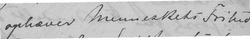ophæver Menneskets Frihed
